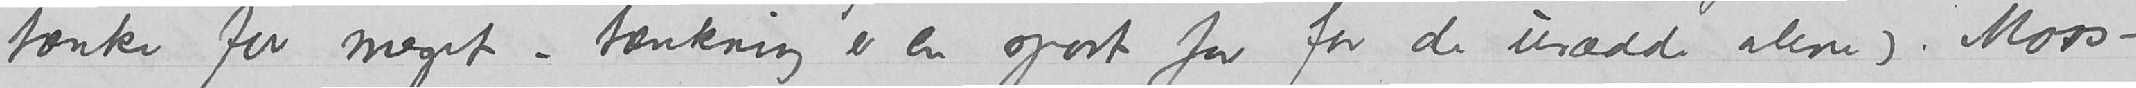faa alle uten de utvalgte fra at tænke for meget – tænkning er en sport for for de urædde alene). Mors-
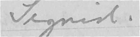Sigrid.
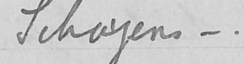Schøyens
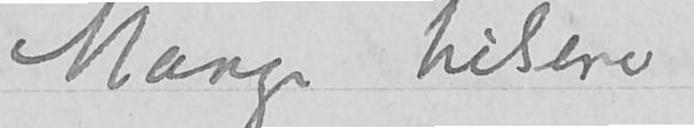Mange Hilsener
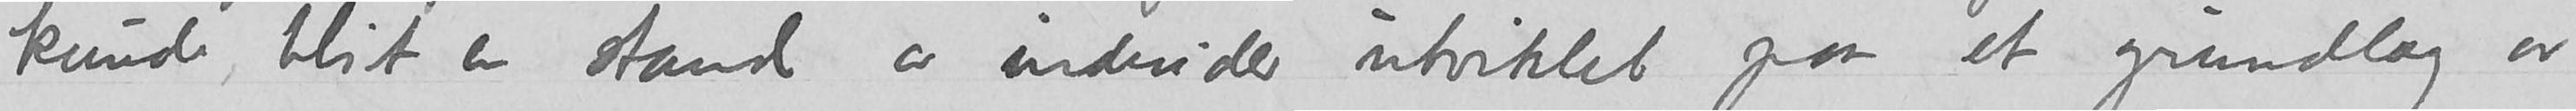kunde blit en stand av individer utviklet paa et grundlag av
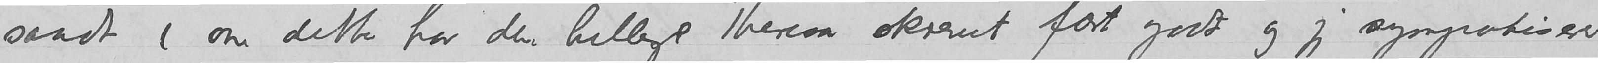sandt (om dette har den hellige Theresa skrevet fært godt og jeg sympatiserer
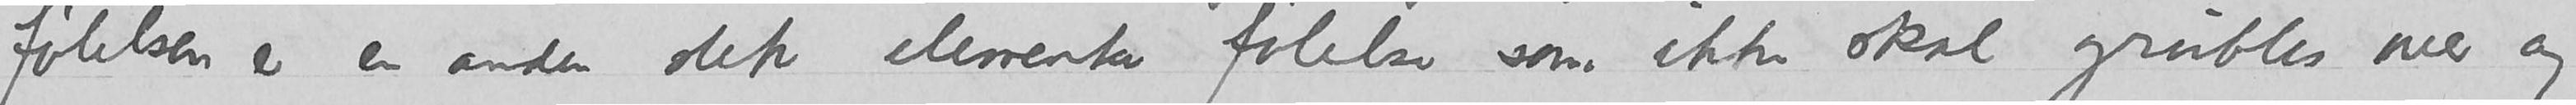følelsen er en anden slik elementær følelse som ikke skal grubles over og
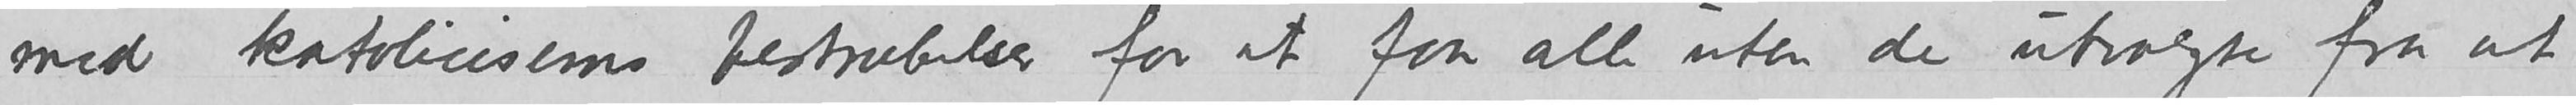med katolicismens bestræbelser for at
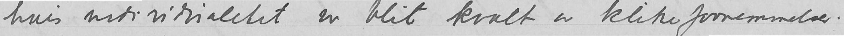hvis individualitet var blit kvalt av klikefornemmelser.
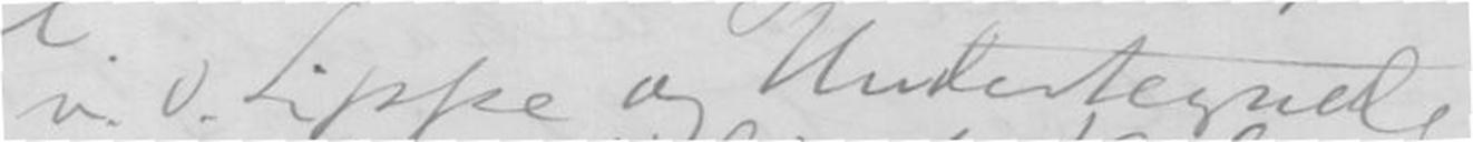v. d. Lippe og Undertegnede
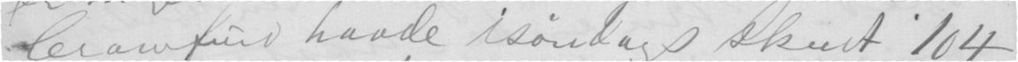Crawford havde isøndags skudt 104
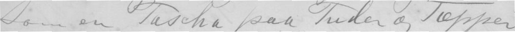som en Pascha paa Puder og Tæpper
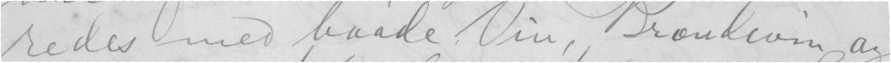redes med baade Vin, Brendevin og
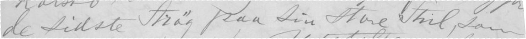det sidste Strøg paa sin store Stril, som
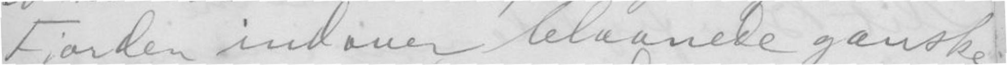Fjorden indover blaanede ganske
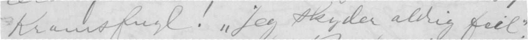Kramfugl! Jeg skyder aldrig feil.
